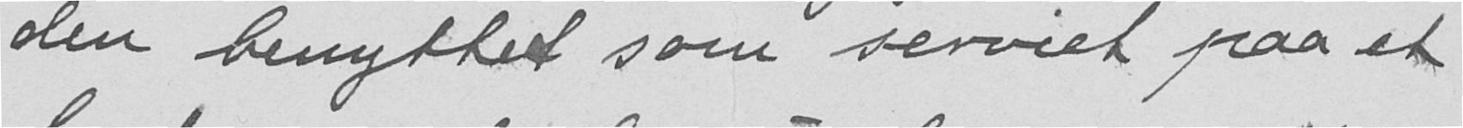den benyttet som serviet paa et
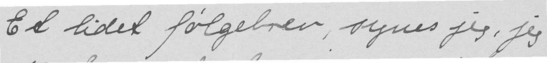Et lidet følgebrev synes jeg, jeg
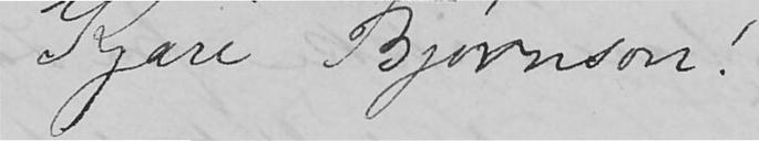Kjære Bjørnson!
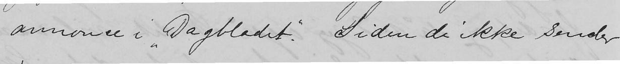annonce i "Dagbladet". Siden de ikke sender
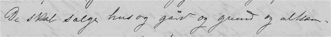De skal sælge hus og gård og grund og altsam-
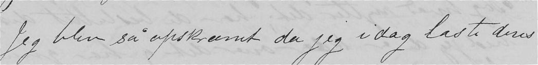Jeg blev så opskræmt da jeg i dag læste deres
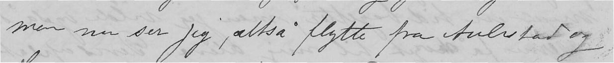men nu ser jeg, altså flytte fra Aulestad og
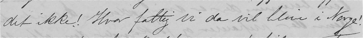det ikke! Hvor fattig vi da vil blive i Norge!
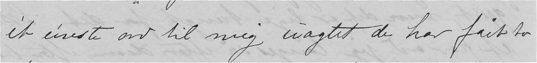ét éneste ord til mig uagtet de har fået to
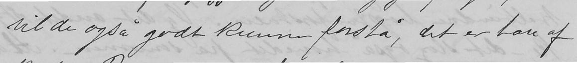vil de også godt kunne forstå, det er bare af
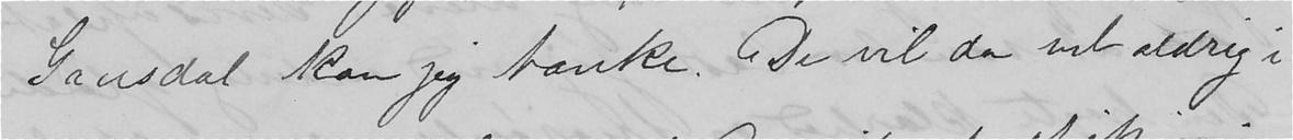Gausdal kan jeg tænke. De vil da vel aldrig i
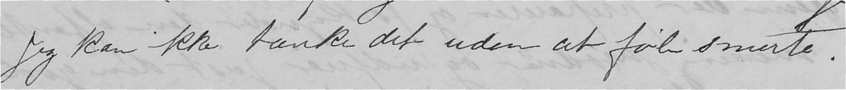Jeg kan ikke tænke det uden at føle smerte.
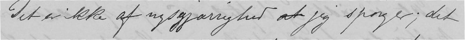Det er ikke af nysgjærrighed at jeg spørger; det
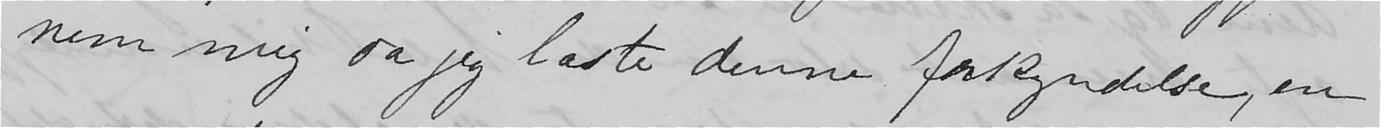nem mig da jeg læste denne forkyndelse, en
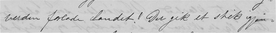verden forlade landet! Der gik et stik igjen-
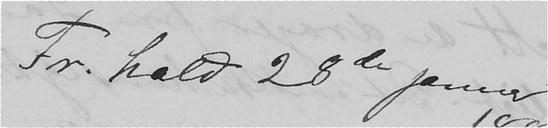Fr. hald 28de januar
